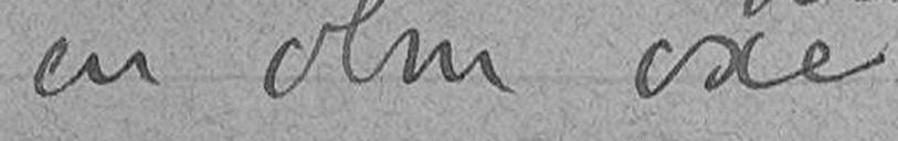en olm oxe
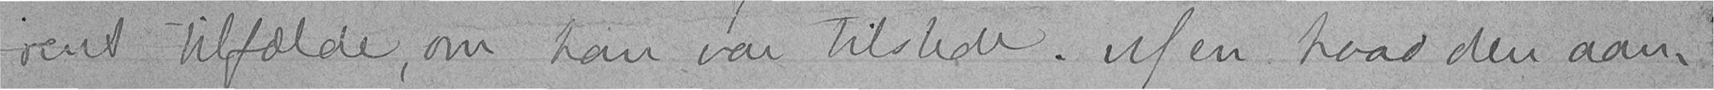rent tilfælde, om han var tilstede. Men hvad den aan-
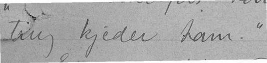ting kjeder ham."
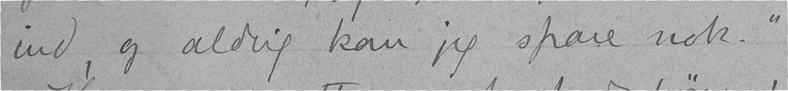ind og aldrig kan jeg spare nok."
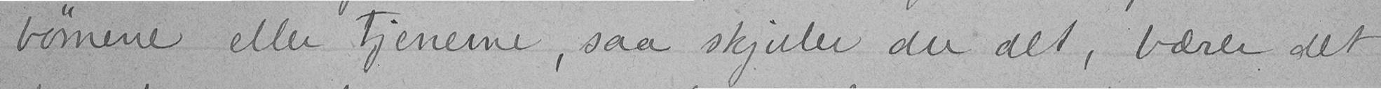børnene eller tjenerne, saa skjuler du alt, bærer det
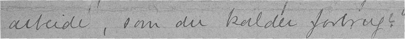arbeide, som du kalder forbrug?"
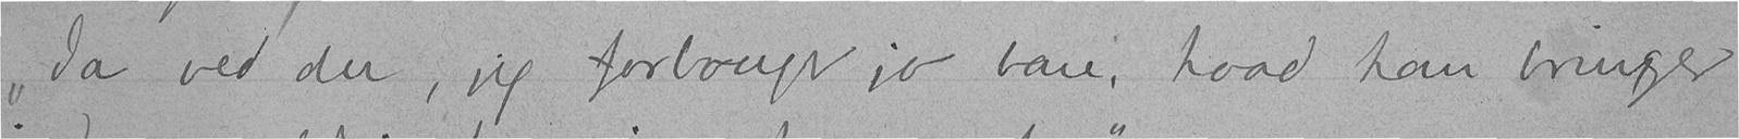"Ja ved du, jeg forbruger jo bare, hvad han bringer
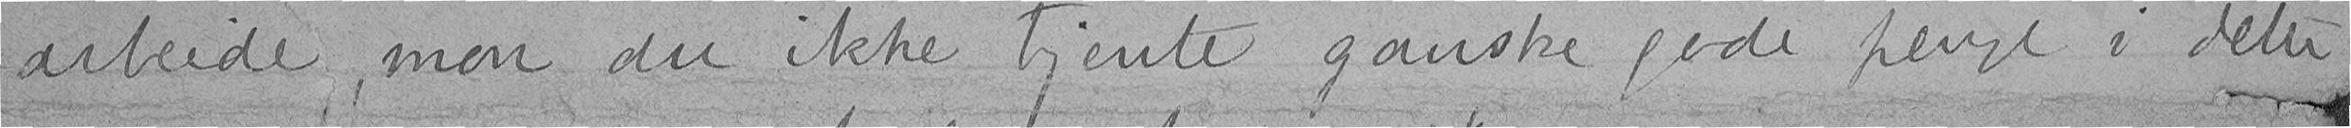arbeide, mon du ikke tjente ganske gode penge i dette
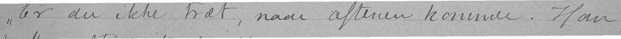"Er du ikke træt, naar aftenen kommer. Han
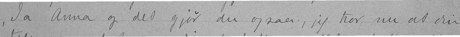"Ja Anna og det gjør du ogsaa, jeg tror nu at din
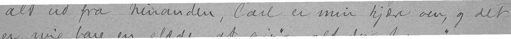alt ud fra hinanden, Carl er min kjære ven, og det
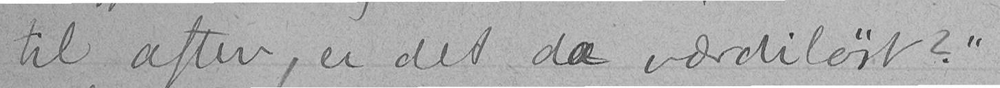til aften, er det da værdiløst?"
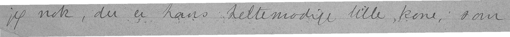jeg nok, du er hans heltemodige lille kone, som
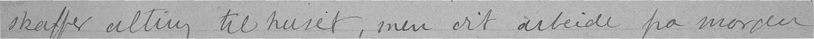skaffer alting til huset, men dit arbeide fra morgen
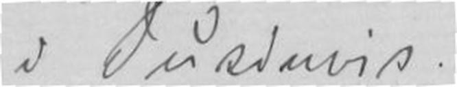i Dusinvis.
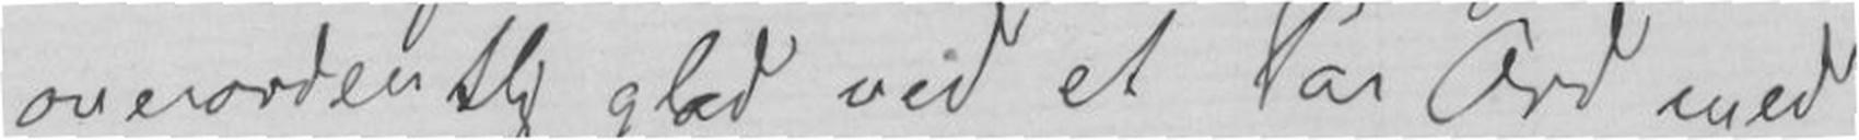overordentlig glad ved et Par Ord med
